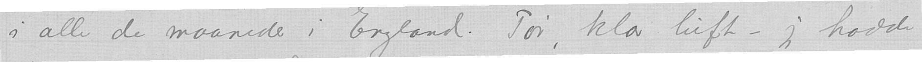i alle de maaneder i England. Tør, klar luft - jeg hadde
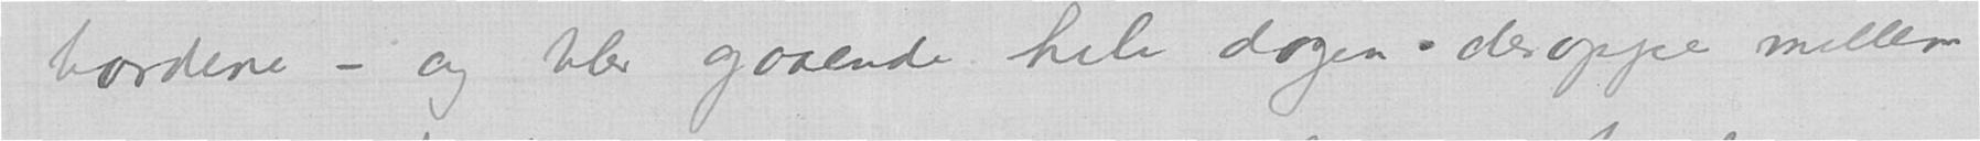bordene - og blev gaaende hele dagen - deroppe mellem
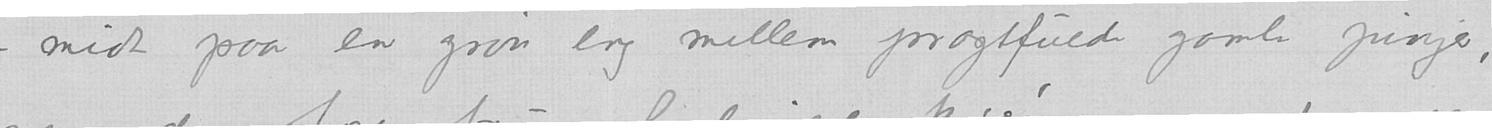- midt paa en grøn eng mellem pragtfulde gamle pinjer,
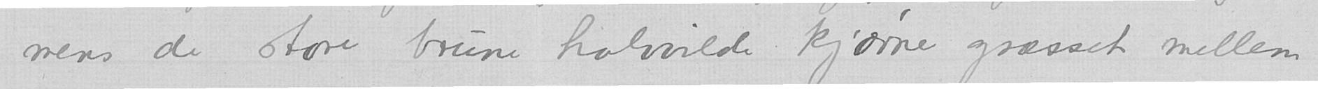mens de store brune halvvilde kjørne græsset mellem
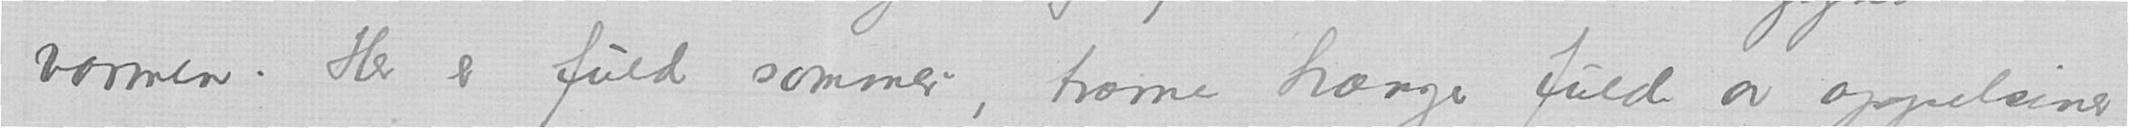varmen. Her er fuld sommer, trærne hænger fulde av appelsiner
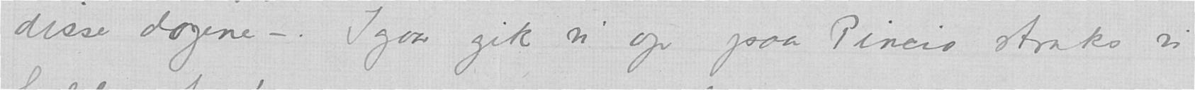disse dagene -. Igaar gik vi op paa Pincio straks vi
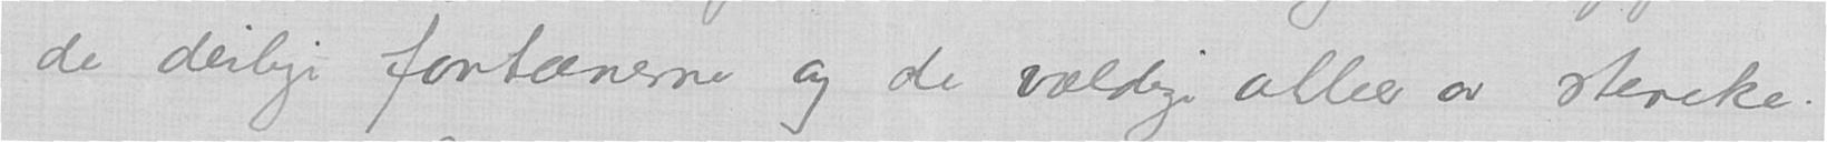de deilige fontænerne og de vældige alleer av steneke. -
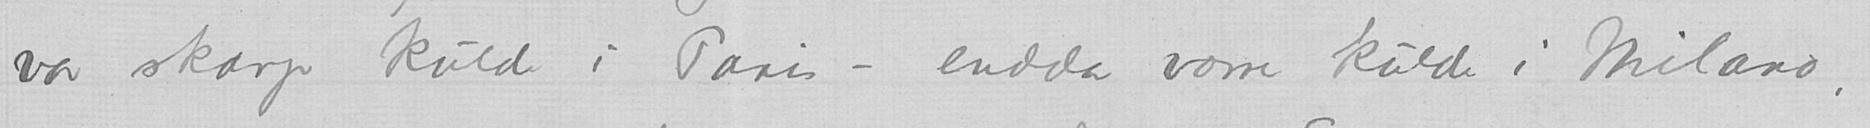var skarp kulde i Paris - endda værre kulde i Milano,
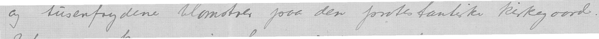og tusenfrydene blomstrer paa den protestantiske kirkegaard.
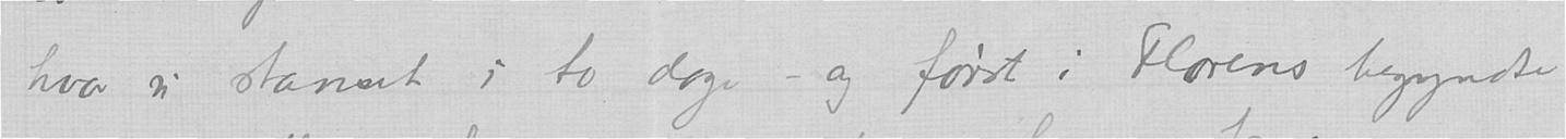hvor vi stanset i to dager - og først i Florens begyndte
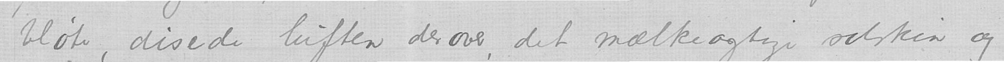bløte, disede luften derover, det mælkeagtige solskin og
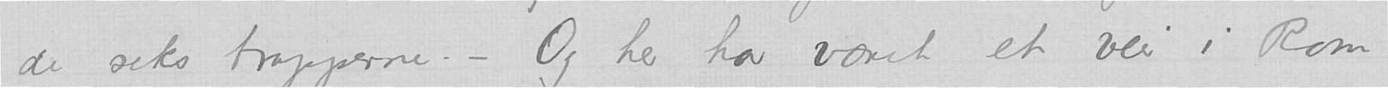de seks trapperne. - Og her har været et veir i Rom
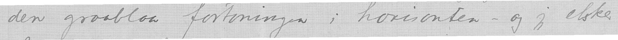den graablaa fortoningen i horisonten - og jeg elsker
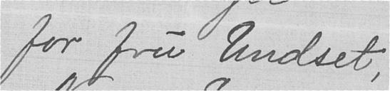for fru Undset,
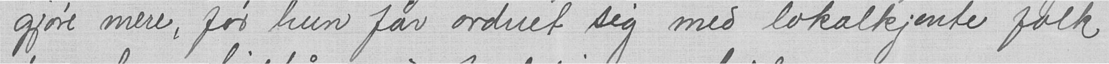gjøre mere, før hun får ordnet sig med lokalkjente folk
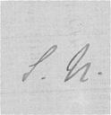S.U.
